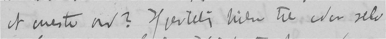et eneste ord? Hjertelig hilsen til eder selv
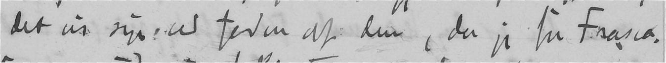det vil sige ved foden af den, da jeg fra Frasca-
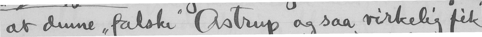at denne "falske" Astrup og saa virkelig fik
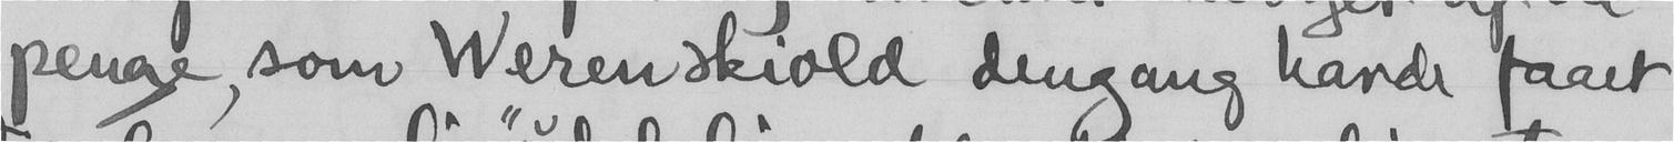penge som Werenskiold dengang havde faaet
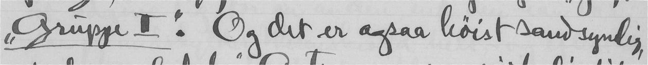"Gruppe 1". Og det er ogsaa høist sandsynlig,
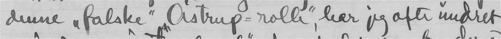denne "falske Astrup-rolle har jeg ofte undret
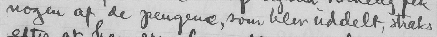nogen af de pengene, som blev uddelt, straks
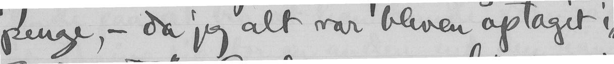penge - da jeg alt var bleven optaget i
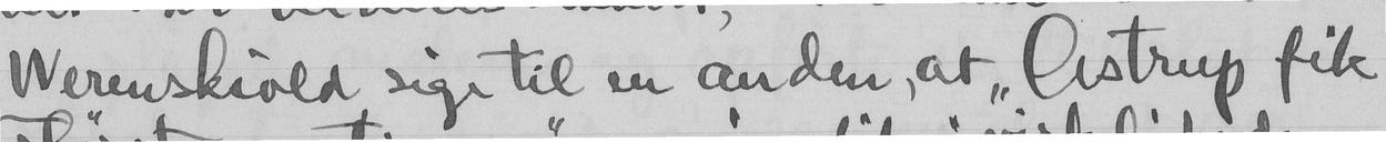Werenskiold sige til en anden, at "Astrup fik
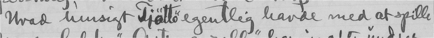Hvad hensigt Tjøtte egentlig havde med at spille
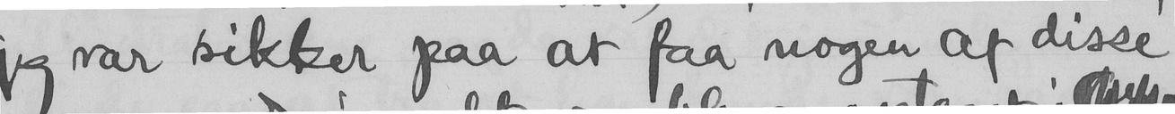jeg var sikker paa at faa nogen af disse
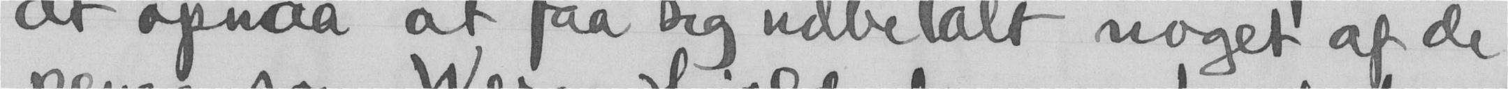at opnaa at faa sig udbetalt noget af de
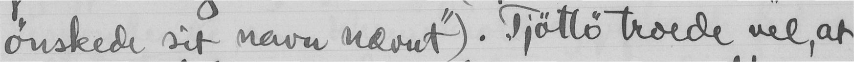ønskede sit navn nævnt"). Tjøttø troede vel, at
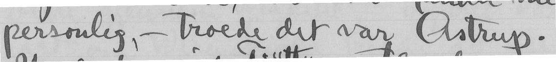personlig, - troede det var Astrup.
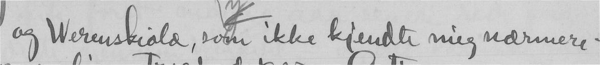og Werenskiold, som ikke kjendte mig nærmere-
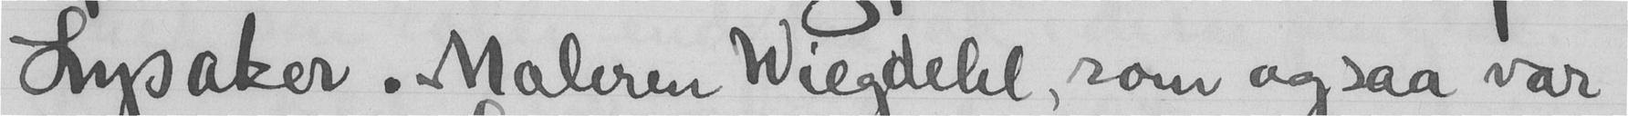Lysaker. Maleren Wiegdehl, som ogsaa var
